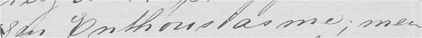gen Enthousiasme; men
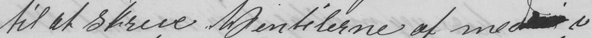til at skrue Ventilerne af med i
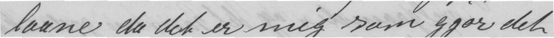laane da det er mig som gjør det
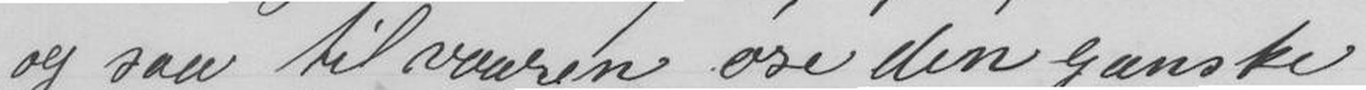og saa til vaaren øse den ganske
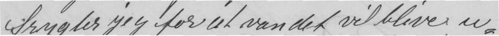frygter jeg for at vandet vil blive u-
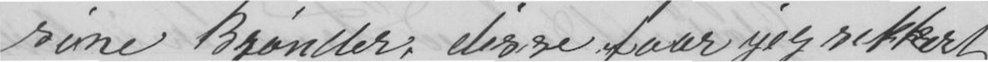sine Brønder, disse faar jeg sikkert
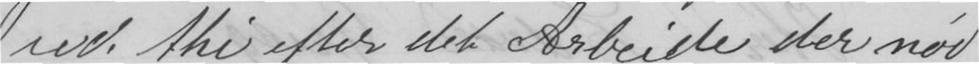ud thi efter det Arbeide der nød-
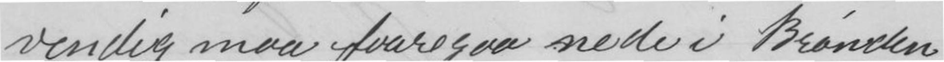vendig maa faaregaa nede i Brønden
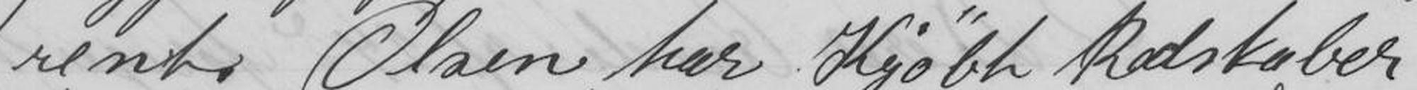rent. Olsen har Kjøbt Redskaber
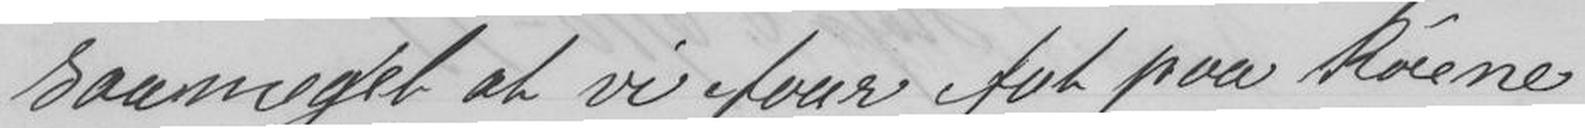saameget at vi faar fat paa Rørene
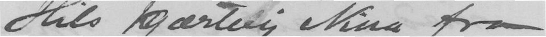Hils hjærtelig Nina fra
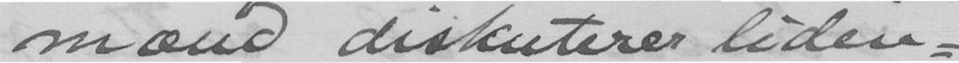mænd diskuterer liden-
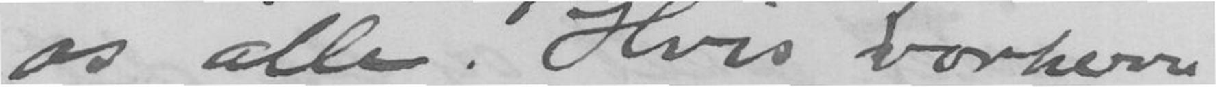os alle. Hvis vorherre
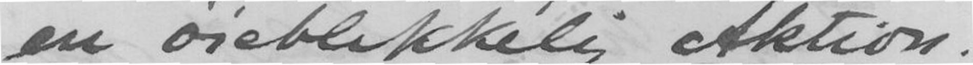en øieblikkelig Aktion.
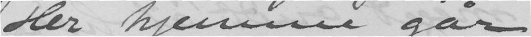Her hjemme går
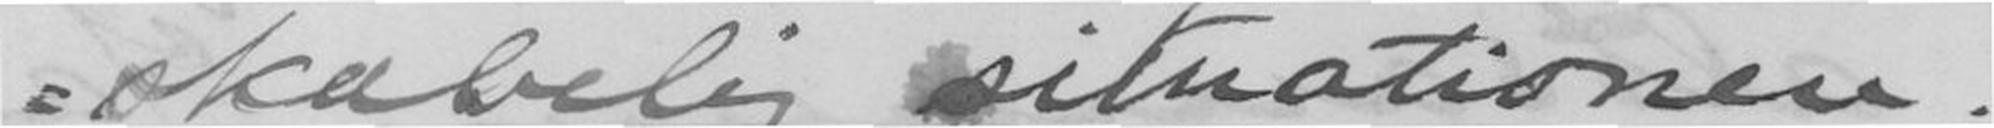skabelig situationen.
